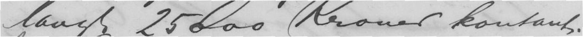langt 25000 Kroner kontant.
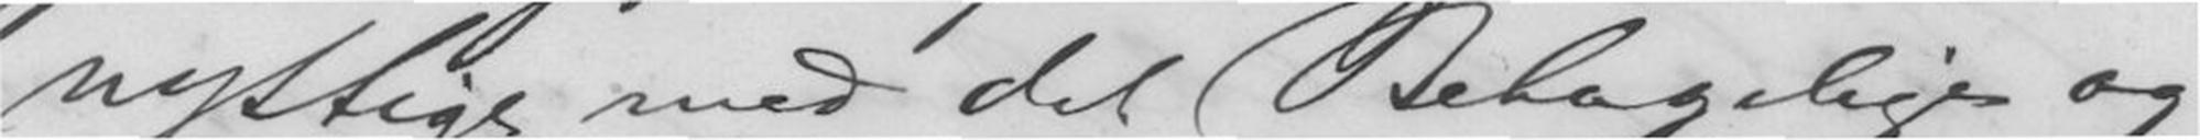nyttige med det Behagelige og
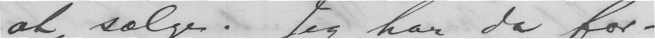at sælge. Jeg har da for-
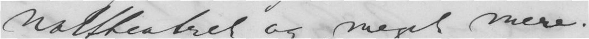naltheatret og meget mere.
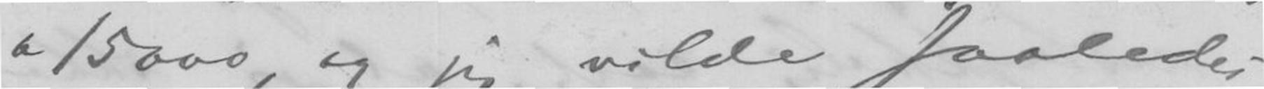a 15000, og jeg vilde saaledes
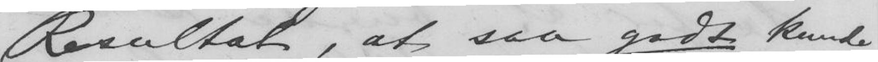Resultat, at saa godt kunde
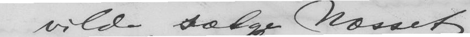jeg vilde sælge Næsset.
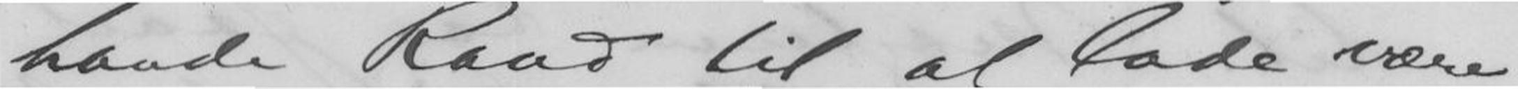havde Raad til at lade være
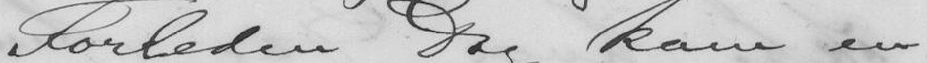Forleden Dag kom en
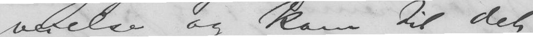veielse og kom til det
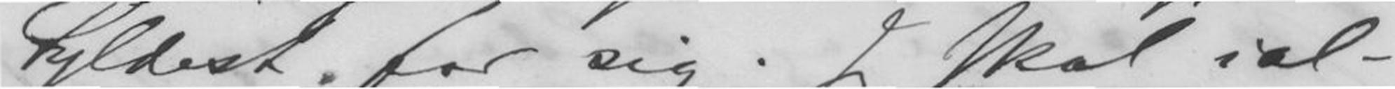Fyldest for sig. Jeg skal ial-
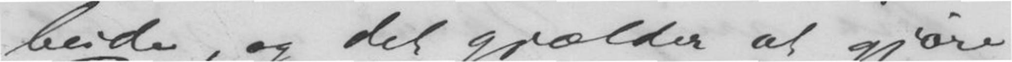beide, og det gjælder at gjøre
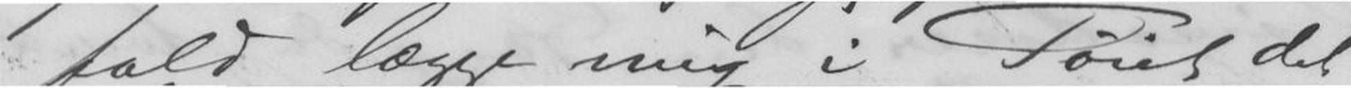fald lægge mig i Tøiet det
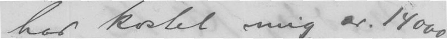Næsset Det har kostet mig ca. 14000
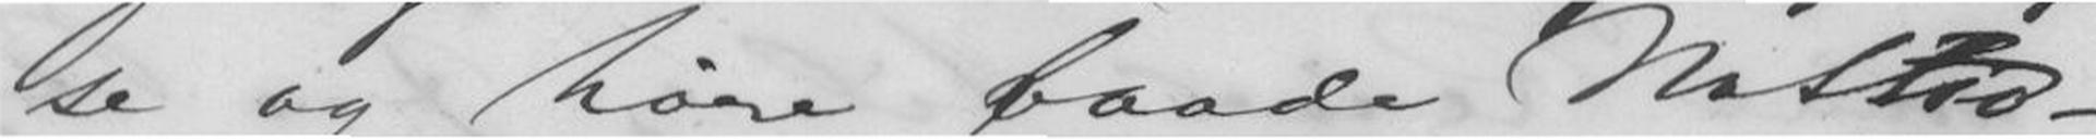se og høre baade Nattio-
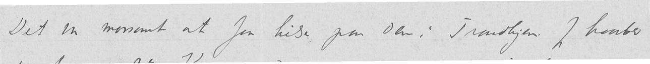Det var morsomt at faa hilse paa Dem i Trondhjem. Jeg haaber
